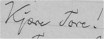Kjære Tore!
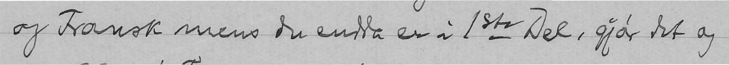og Fransk mens du endda er i 1ste Del, gjør det og
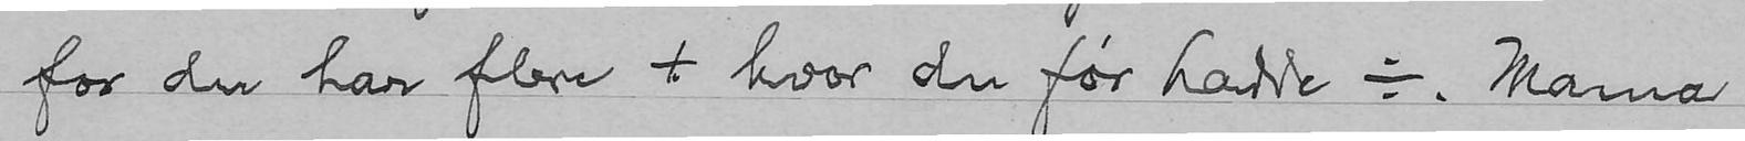for du har flere + hvor du før hadde ÷. Mama
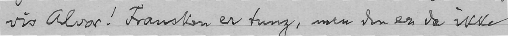vis Alvor! Fransken er tung, men den er da ikke
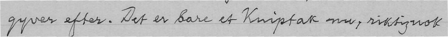gyver efter. Det er bare et Kniptak nu, riktignok
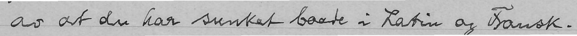av at du har sunket baade i Latin og Fransk.
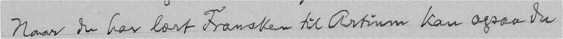Naar du har lært Fransken til Artium kan ogsaa du
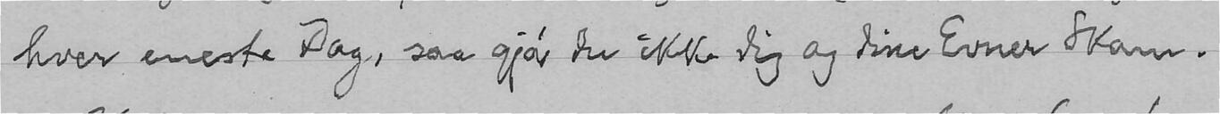hver eneste Dag, saa gjør du ikke dig og dine Evner Skam.
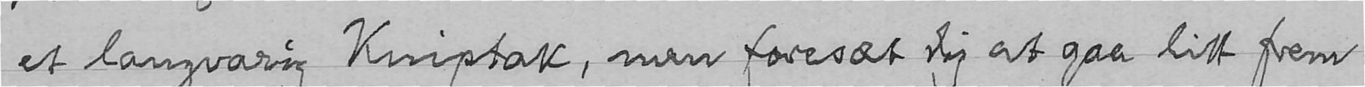et langvarig Kniptak, men foresæt dig at gaa litt frem
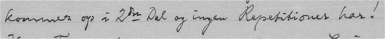kommer op i 2den Del og ingen Repetitioner har!
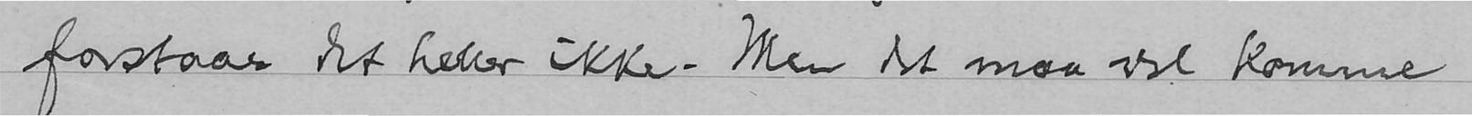forstaar det heller ikke. Men det maa vel komme
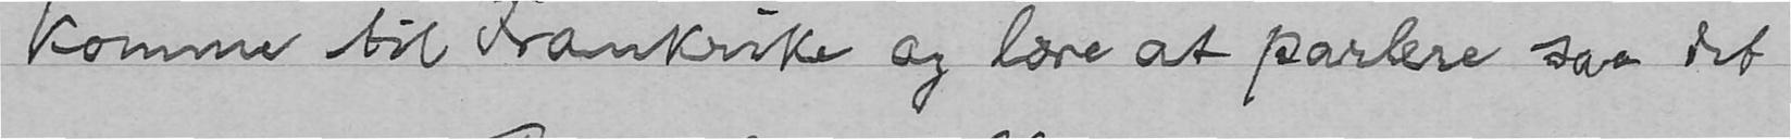komme til Frankrike og lære at parlere saa det
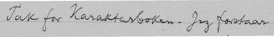Tak for Karakterboken. Jeg forstaar
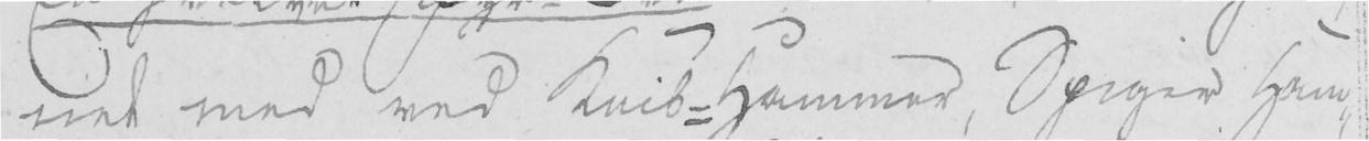net med ved Knib-Hammer, Spiger Ham-
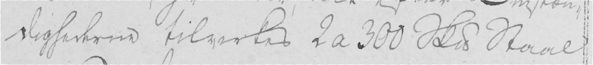dighederne tilvirkes 2 a 300 Skpd Staal
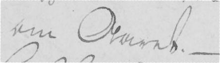om Aaret. -
